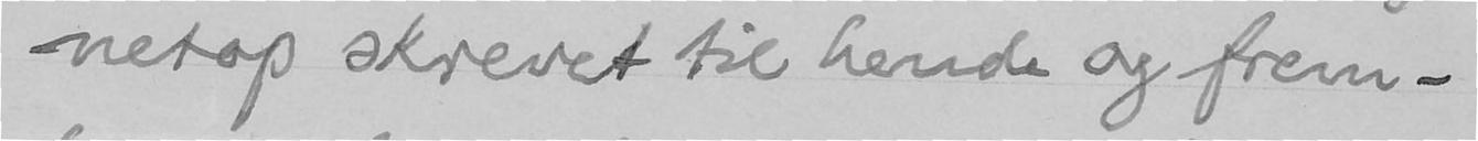netop skrevet til hende og frem-
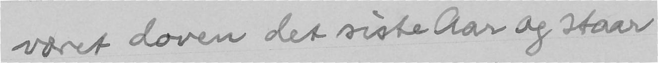været doven det siste Aar og staar
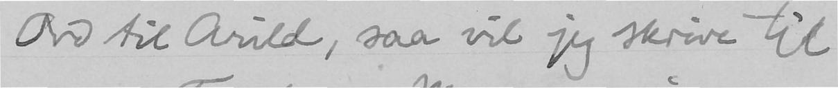Ord til Arild, saa vil jeg skrive til
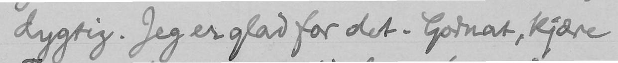dygtig. Jeg er glad for det. Godnat, kjære
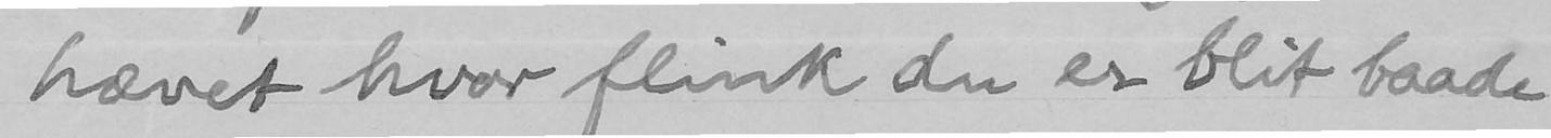hævet hvor flink du er blit baade
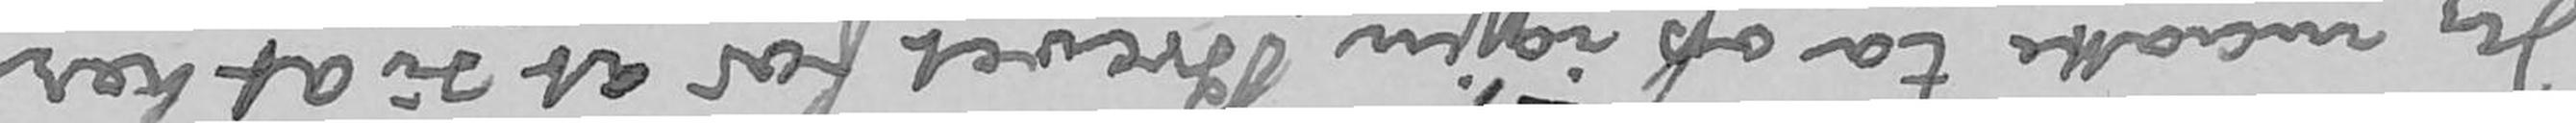Jeg maatte ta op igjen Brevet for at si at her
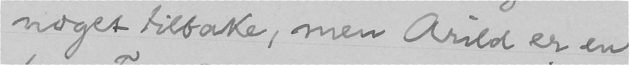noget tilbake, men Arild er en
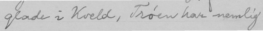glade i Kveld, Trøen har nemlig
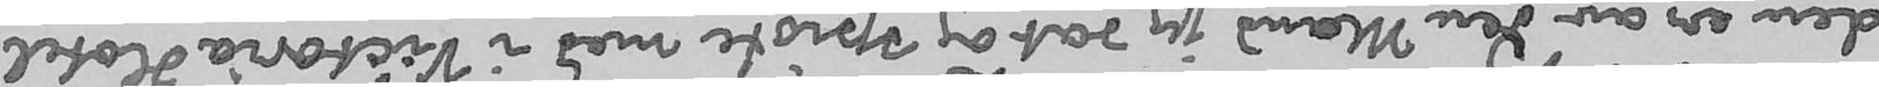den er av den Mand jeg sat og spiste med i Victoria Hotel
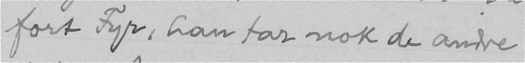fort Fyr, han tar nok de andre
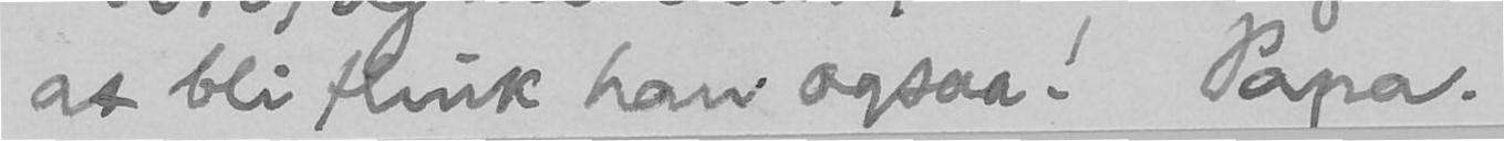at bli flink han ogsaa! Papa.
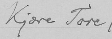Kjære Tore,
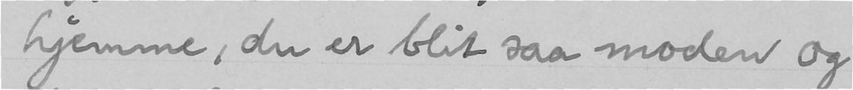hjemme, du er blit saa moden og
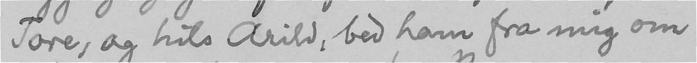Tore, og hils Arild, bed ham fra mig om
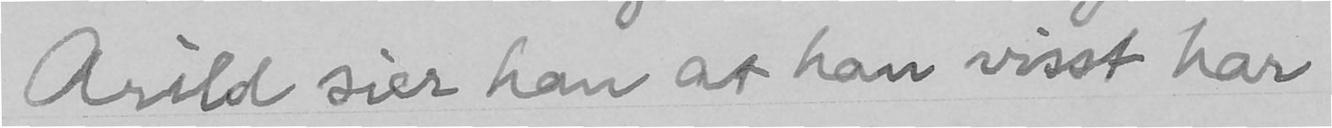Arild sier han at han visst har
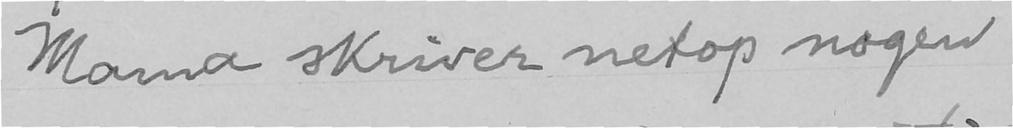Mama skriver netop nogen
